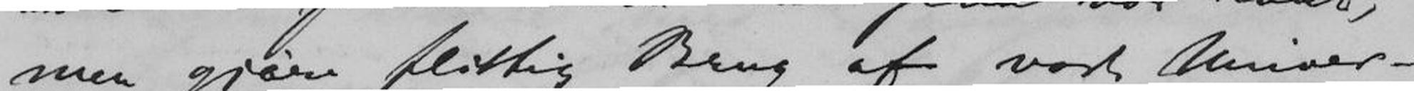men gjøre flittig Brug af vort Univer-
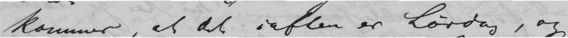kommer, at det iaften er Lørdag, og
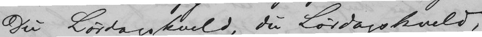Du Lørdagskveld, du Lørdagskveld,
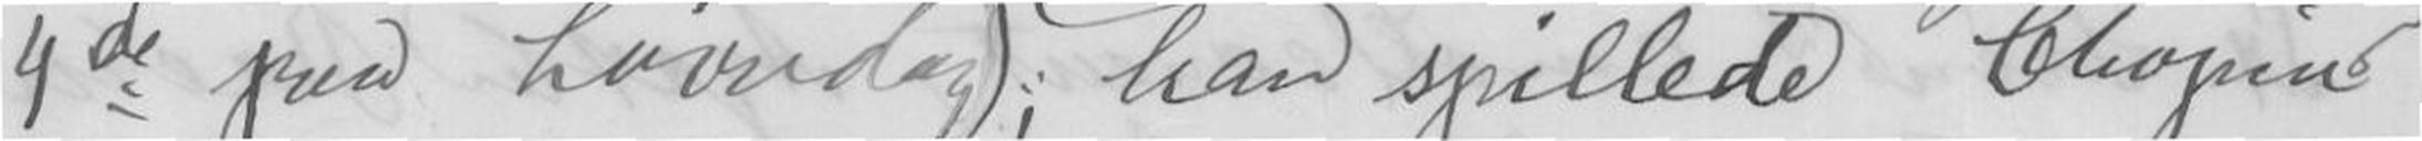4de paa Løverdag), han spillede Chopin
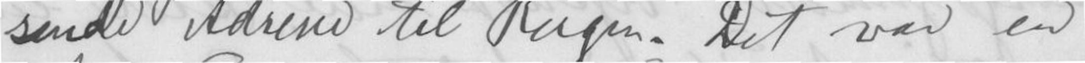sende Adresse til Bergen. Det var en
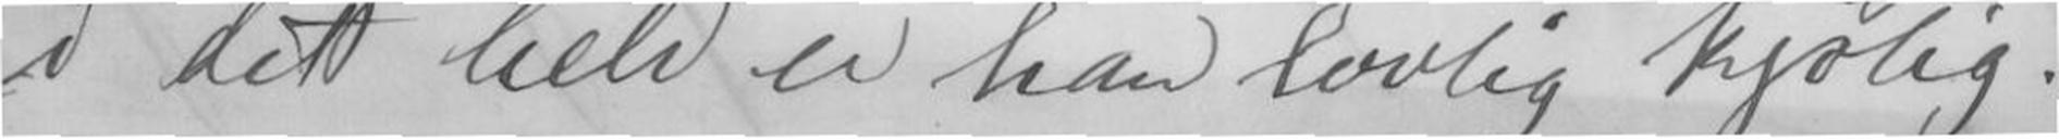i det hele er han lovlig kjølig
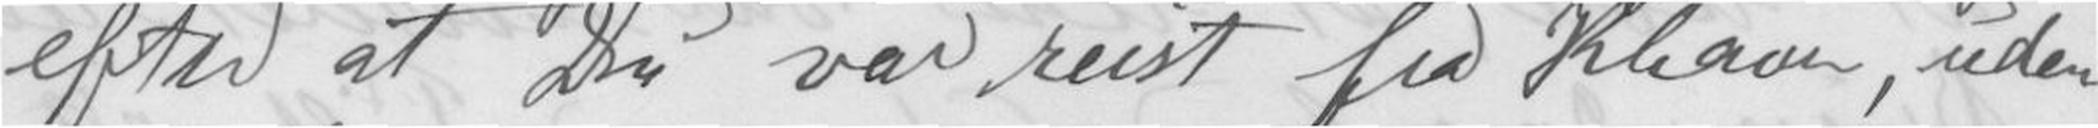efter at  Du var reist fra Khavn, uden
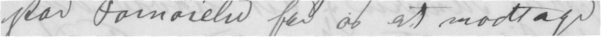stor Fornøielse for os at modtage
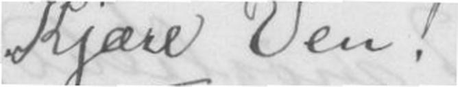Kjære Ven!
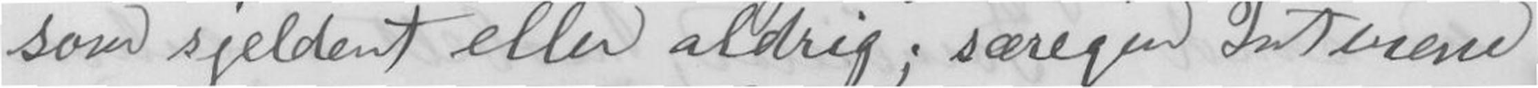som sjeldent eller aldrig; særegen Interesse
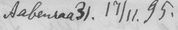Aabenraa 31. 17/11.95.
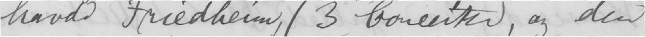havde Friedheim (3 Concerter, og den
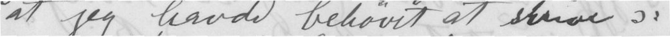at jeg havde behøvet at skrive ɔ:
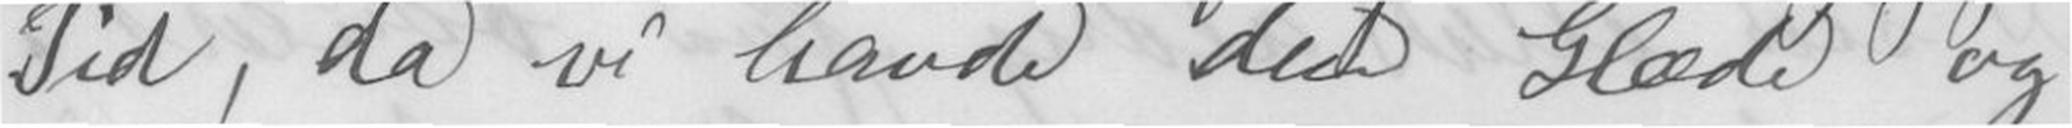Tid, da vi havde den Glæde og
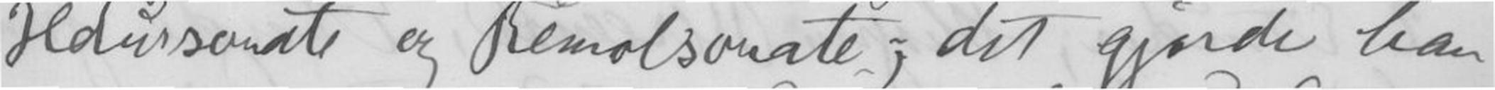Hdursonate og Bemolsonate; det gjorde han
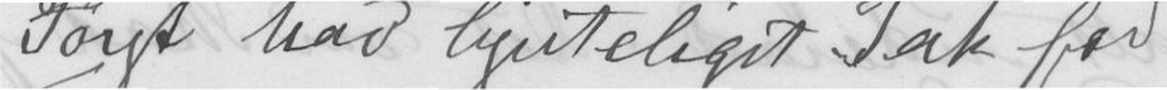Først hav hjerteligst Tak for
